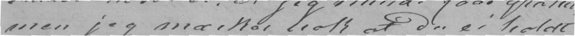men jeg mærker nok at Du ei holdt
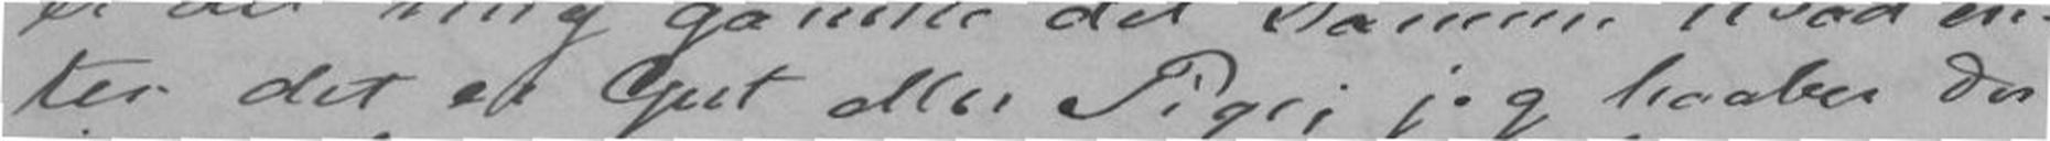ten det er Gut eller Pige; jeg haaber Du
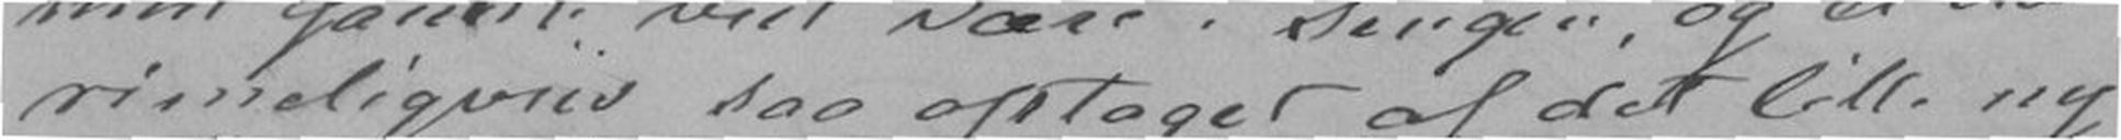rimeligvis saa optaget af det lille ny
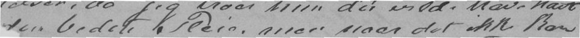den bedste Pleie, men naar det ikke kan
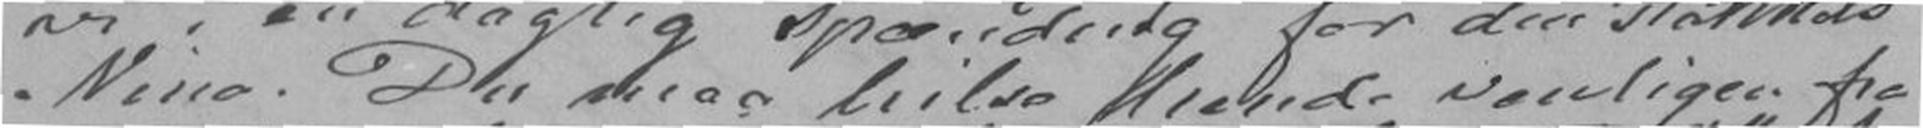Nina. Du maa hilse hende venligen fra
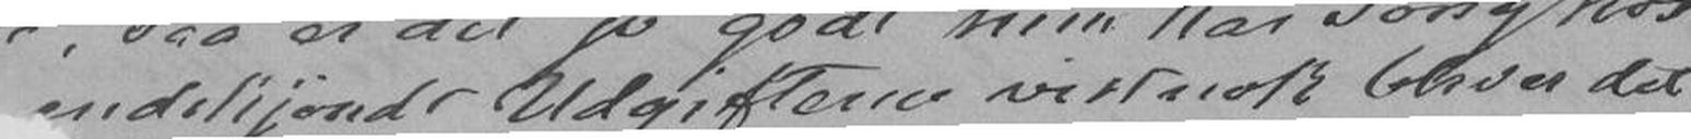endskjøndt Udgifterne vistnok bliver det
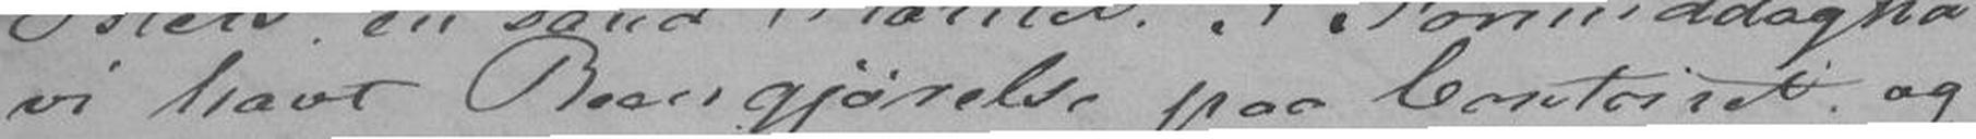vi havt Reengjørelse paa Contoiret og
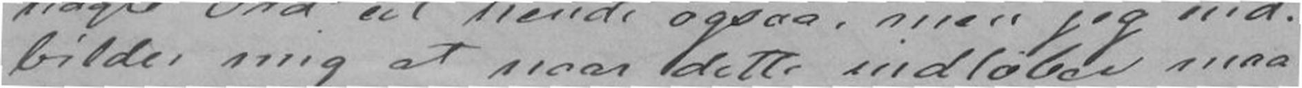bilder mig at naar dette indløber maa
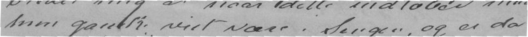hun ganske vist være i Sengen, og er da
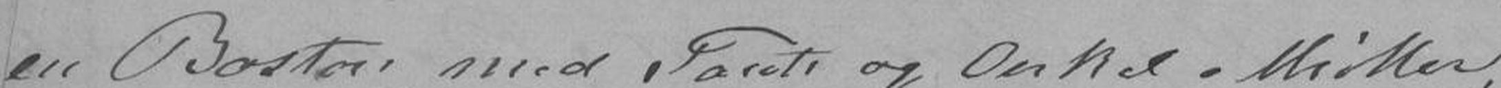en Boston med Tante og Onkel Møller,
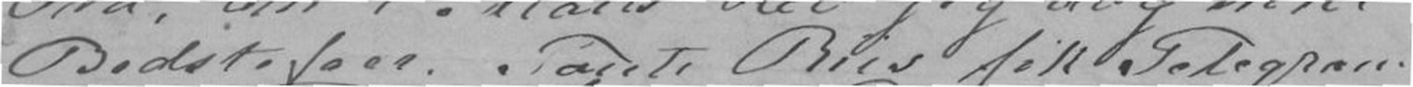Bedstefaer. Tante Riis fik Telegram
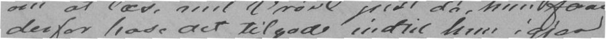derfor have det tilgode indtil hun igjen
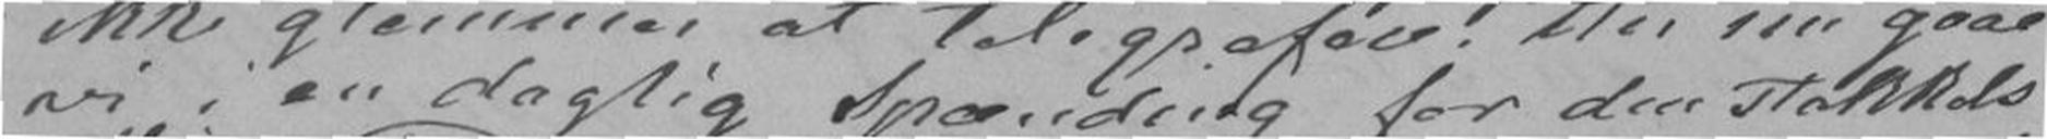vi i en daglig Spænding for den stakkels
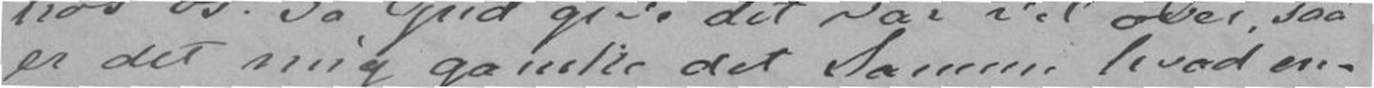er det mig ganske det Samme hvad en-
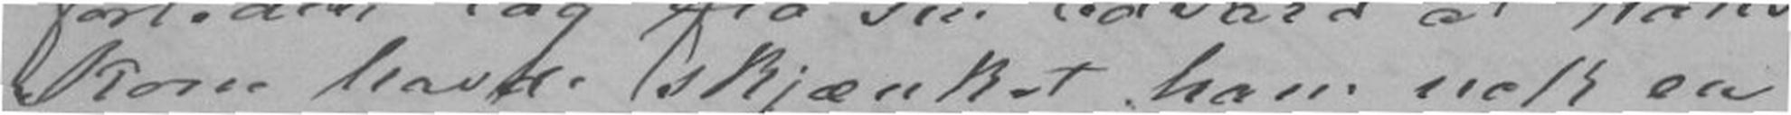Kone havde skjænket ham nok en
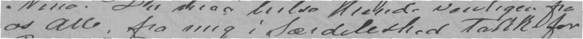os Alle, fra mig i Særdeleshed takk for
